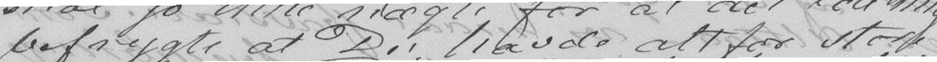befrygte at Du havde altfor store
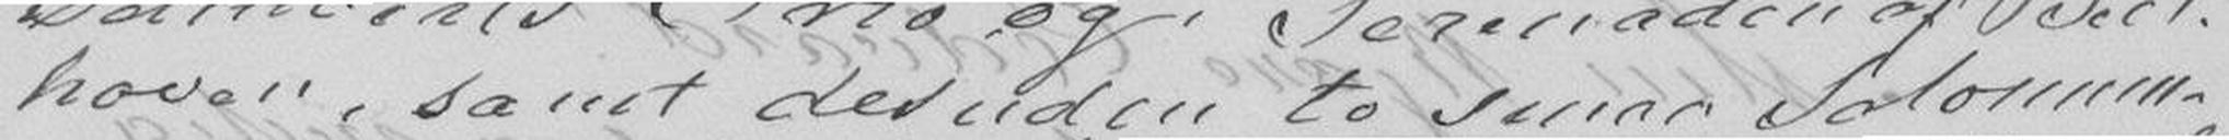hoven, samt desuden to smaa Solonum-
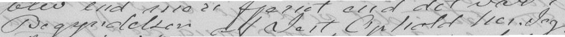Begynddelsen af Jert Ophold her, Jeg
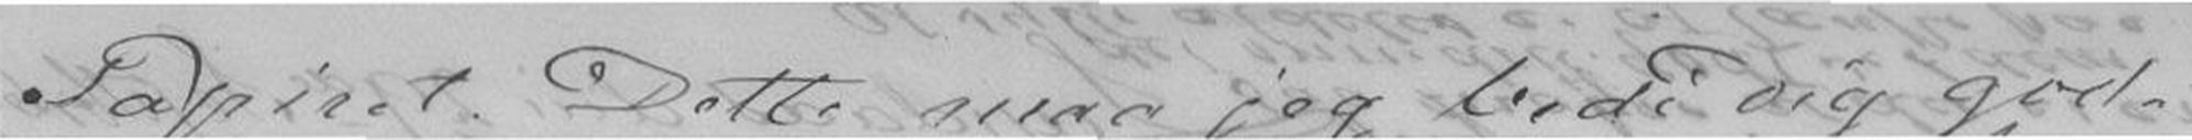Papiret. Dette maa jeg bede Dig god-
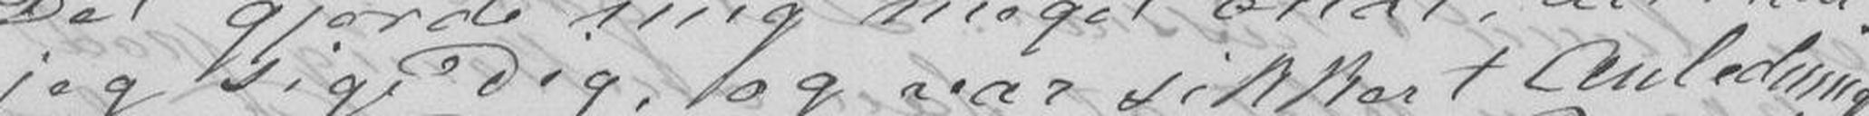jeg sige Dig, og var sikkert Anledning
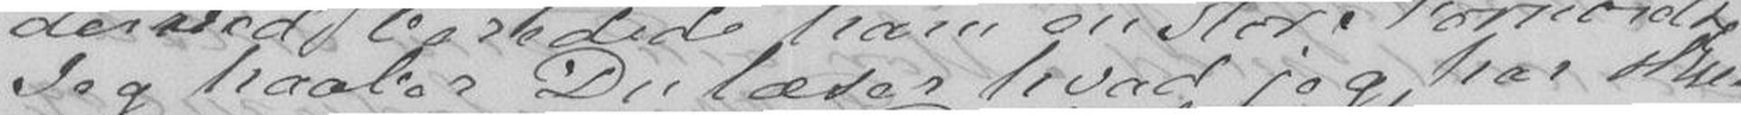Jeg haaber Du læser hvad jeg har skre-
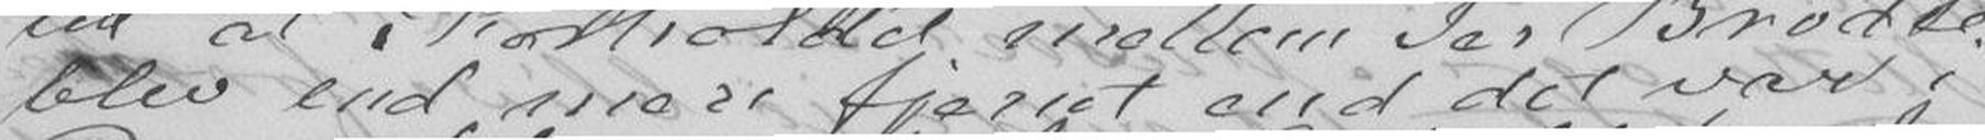blev end mere fjernt end det var i
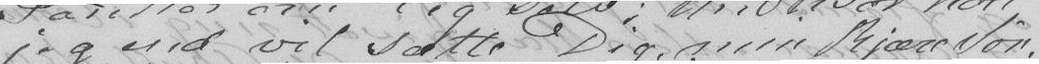jeg end vil sætte Dig, min kjære Søn.
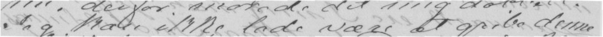Jeg kan ikke lade være at gribe denne
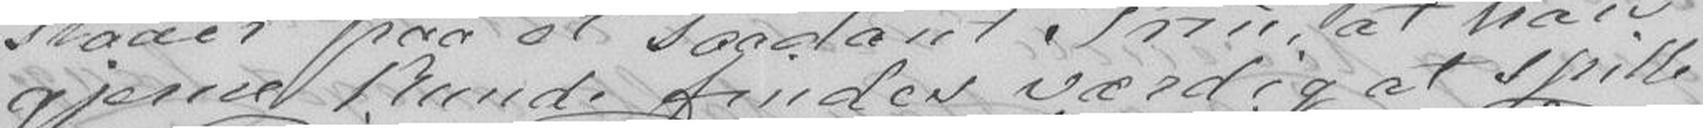staaer paa et saadant Trin, at spille
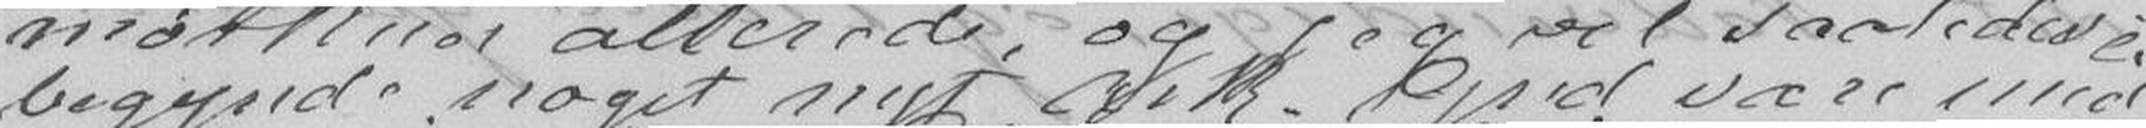begynde noget nyt Ark; Gud være med
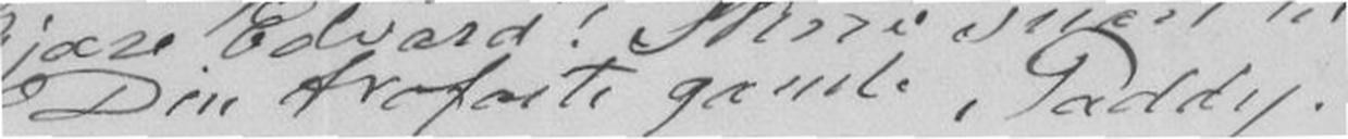Din trofaste gamle Paddy.
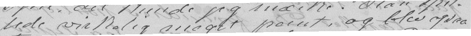lede virkelig meget peent, og blev ogsaa
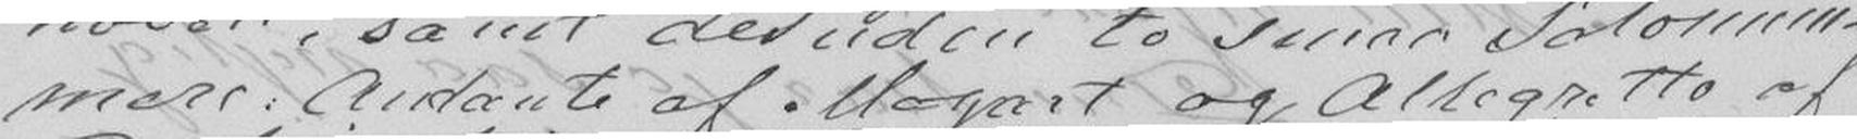mere: Andante af Mozart og Allegretto af
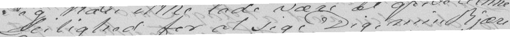Leilighed for at sige Dig, min kjære
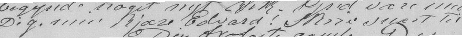Dig min kjære Edvard! Skriv snart til
